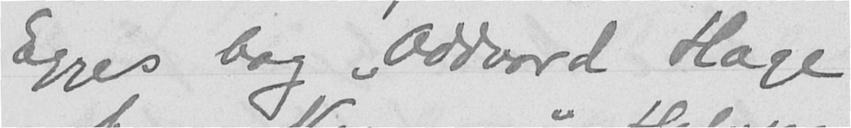Egges bog "Oddvard Hage
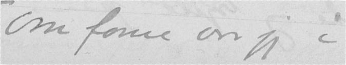Om form var jeg i
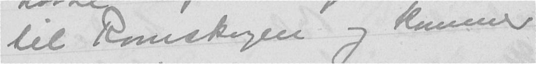til Romskogen og kommer
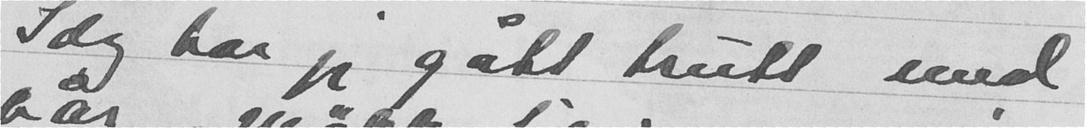Idag har jeg gått trutt med
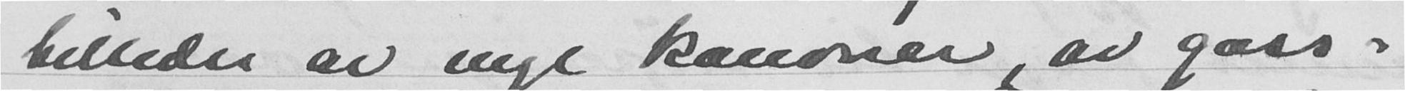billeder av nye kanoner, av gass-
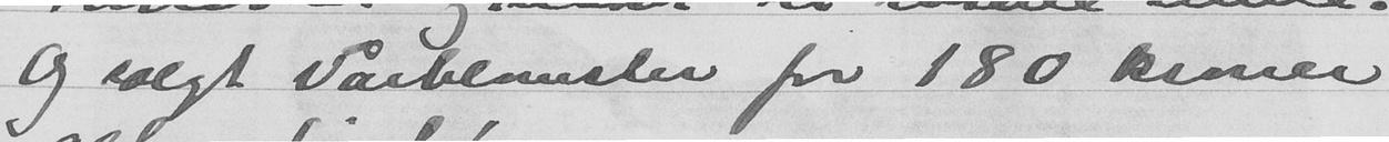Og solgt Vårblomster for 180 kroner
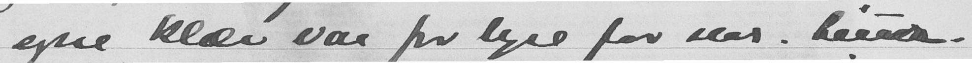egne klær var for lyse for nas ten.
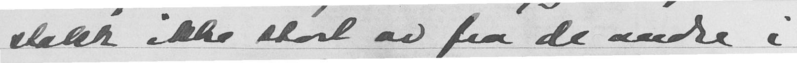stakk ikke stort av fra de andre i
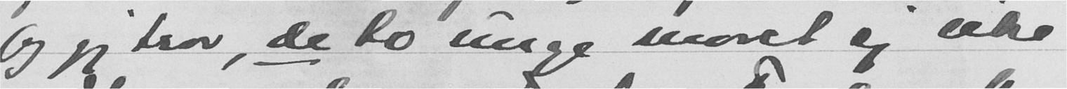Og jeg tror, de to unge moret sig like
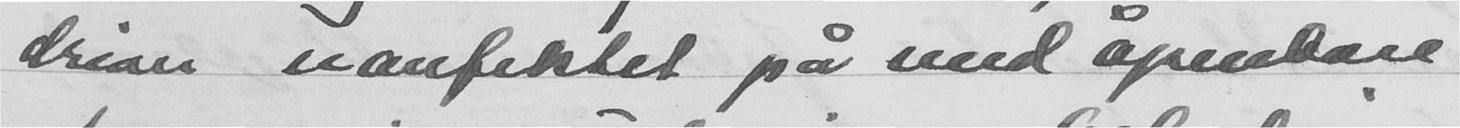driver uanfektet på med åpenbare
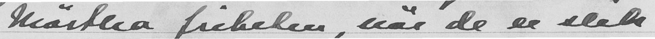Märtha friheten, når de er slik
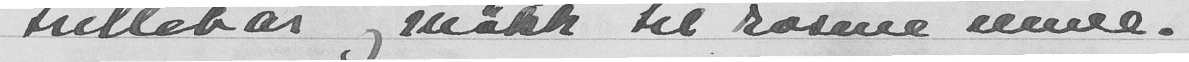trillebår og møkk til rosene mine.
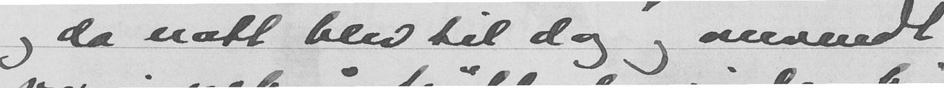og da natt blev til dag og omvendt
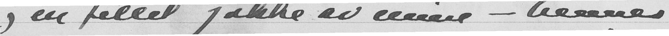og en fillet jakke av mine - hennes
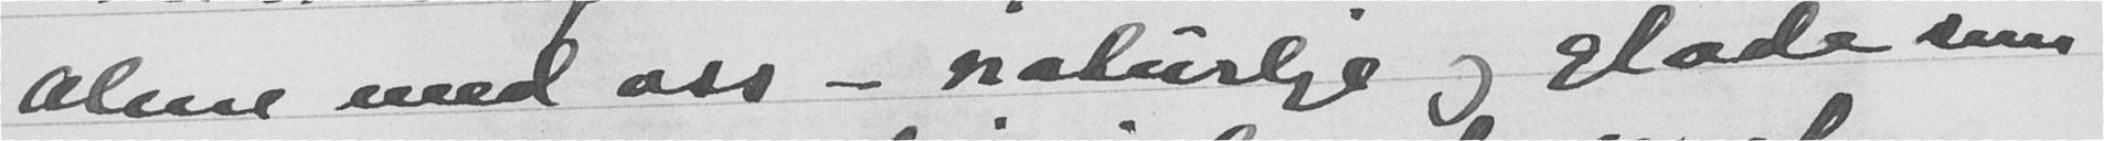alene med oss - naturlige og glade som
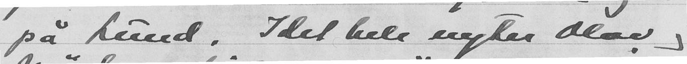på kund. Idet hele nyter Olav og
